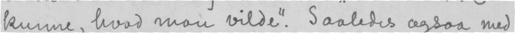kunne, hvad man vilde. Saaledes ogsaa med
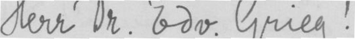Herr Dr. Edv. Grieg!
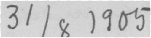31/8 1905.
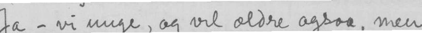Ja - vi unge, og vel ældre ogsaa, men
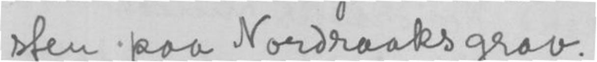sten paa Nordraachs grav.
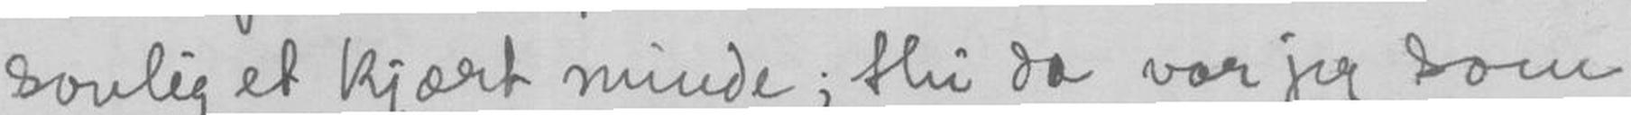sonlig et kjært minde; thi da var jeg som
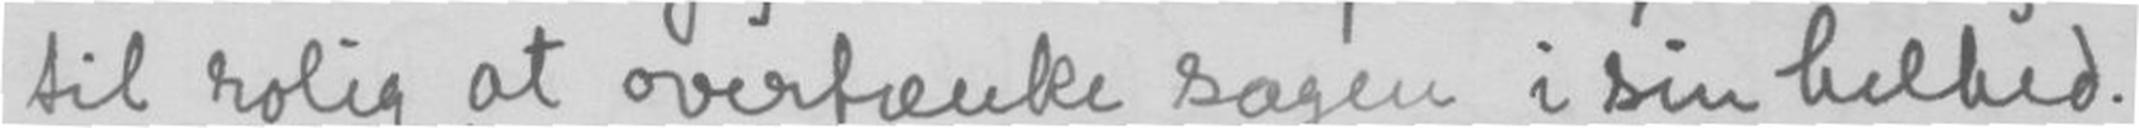til rolig at overtænke sagen i sin helhed.
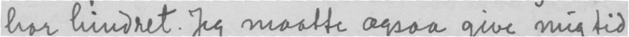har hindret. Jeg maatte ogsaa give mig tid
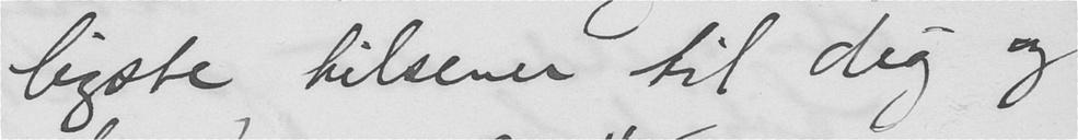ligste hilsener til dig og
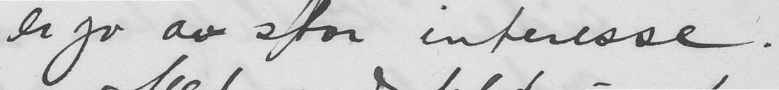er jo av stor interesse. -
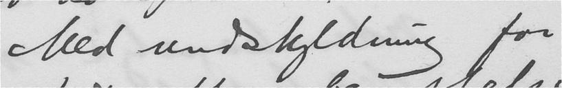Med undskyldning for
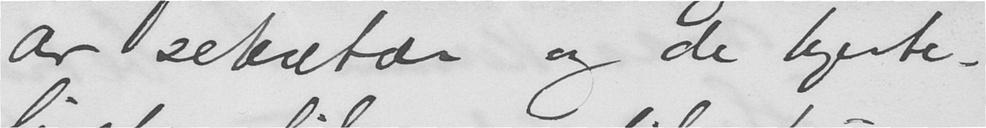av sekretær og de hjerte-
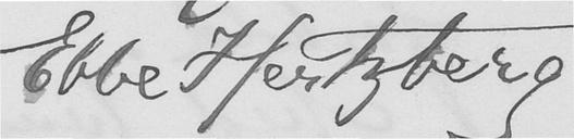Ebbe Hertzberg.
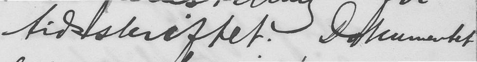tidsskriftet. Dokumentet
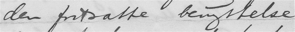den fortsatte benyttelse
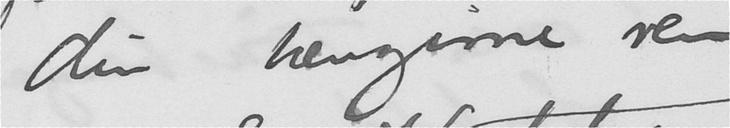din hengivne ven
